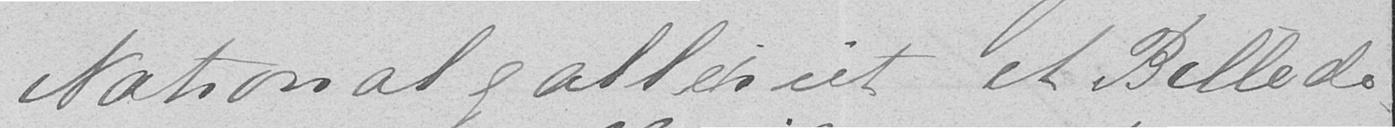Nationalgalleriet et Billede,
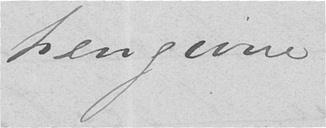hengivne
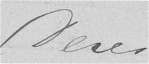Deres
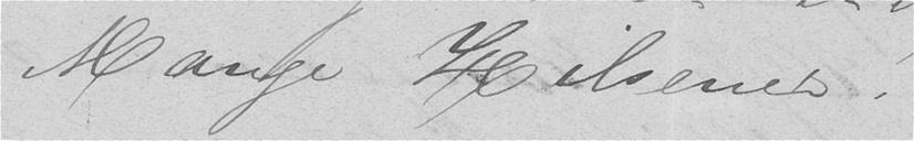Mange Hilsener!
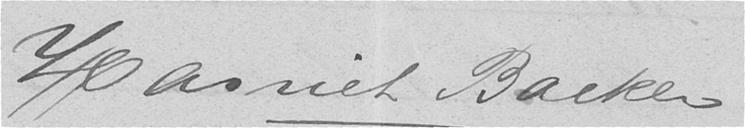Harriet Backer
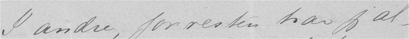I andre, forresten har jeg al-
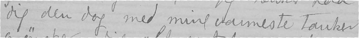dig den dag med mine varmeste tanker
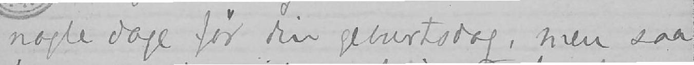nogle dage før din geburtsdag, men saa
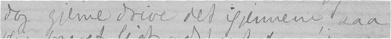dog gjerne drive det igjennem, saa
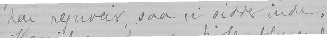har regnveir, saa vi sidder inde.
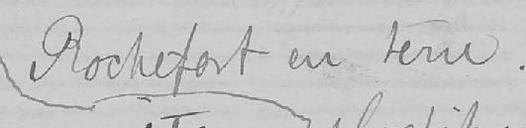Rochefort en terre.
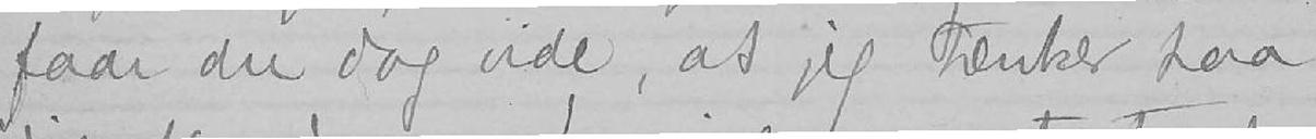faar du dog vide, at jeg tænker paa
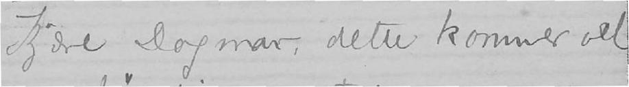Kjære Dagmar, dette kommer vel
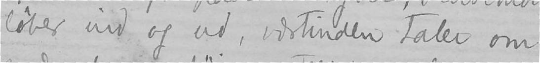løber ind og ud, værtinden taler om
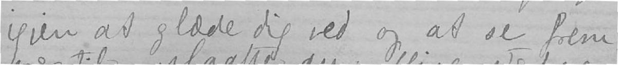igjen at glæde dig ved og at se frem-
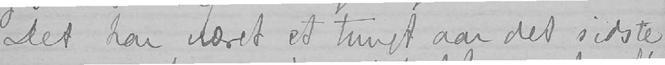Det har været et tungt aar det sidste,
